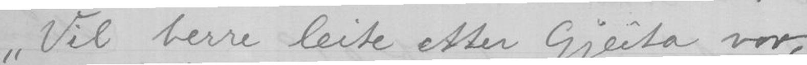"Vil berre leite etter Gjeita vor,
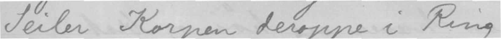Seiler Korpen deroppe i Ring,
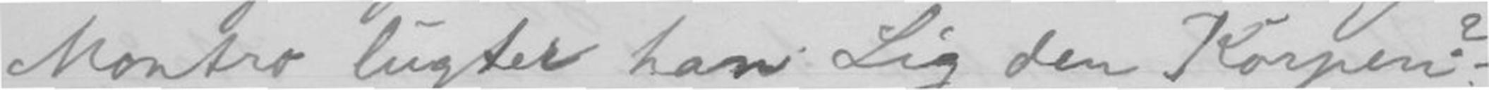Montro lugter han Lig den Korpen?
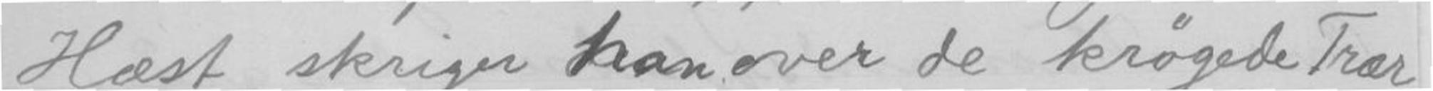Hæst skriger han over de krøgede Trær
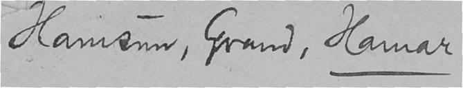Hamsun, Grand, Hamar
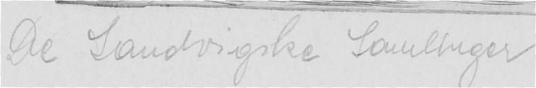"De Sandvigske Samlinger"
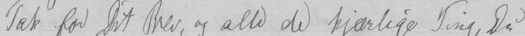Tak for Dit Brev, og alle de kjærlige Ting, Du
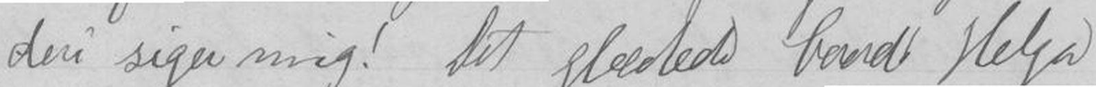deri siger mig! Det glædede baade Helga
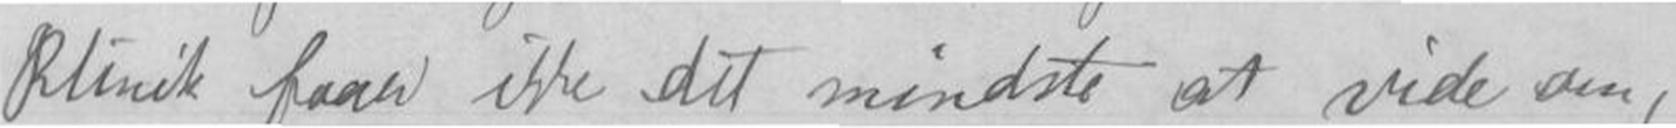klinik faaer ikke det mindste at vide om,
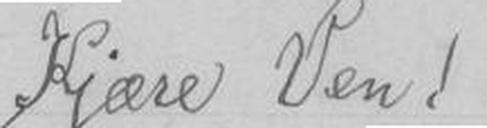Kjære Ven!
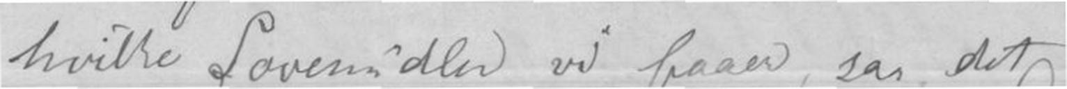hvilke Sovemidler vi faaer, saa det
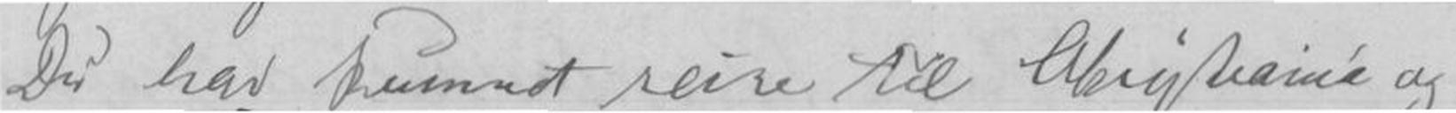Du har kunnet reise til Christiania og
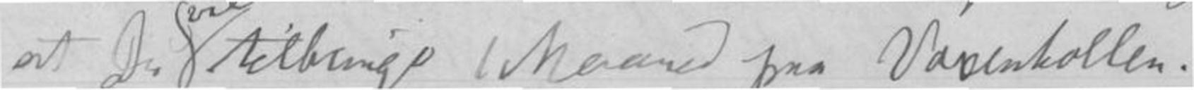at Su vil tilbringe 1 Maaned paa Voksenkollen.
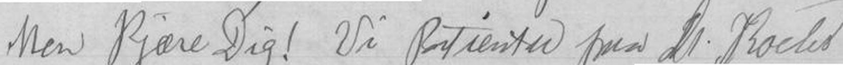Men kjære Dig! Vi Pasienter paa Dr. Rochs
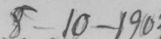8-10-1903
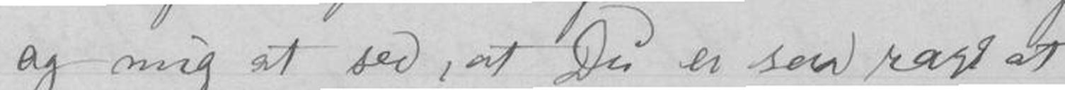og mig at see, at Du er saa rask at
